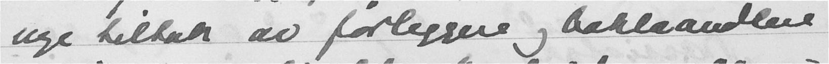nye tiltak av forleggere og bokhandlere
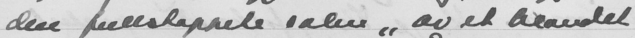den fullstappete salen, "av et blandet
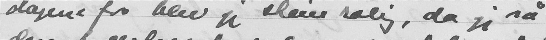dagene før blev jeg stein rolig, da jeg så
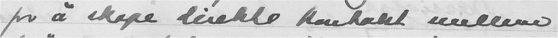for å skape direkte kontakt mellom
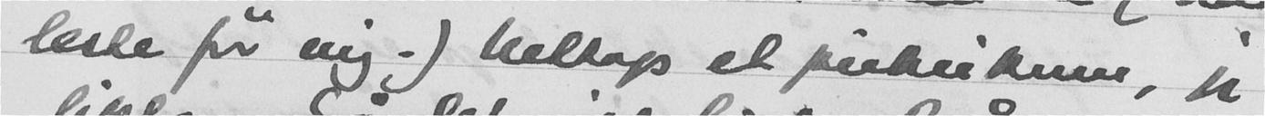leste for sig.) Heltops et puklikum, jeg
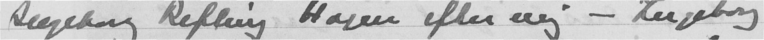Ingeborg Refling Hagen efter mig - Ingeborg
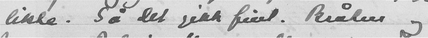likte. Så det gikk fint. Bråten og
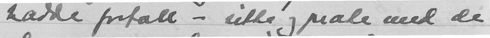hadde forfall - sitte og prate med de
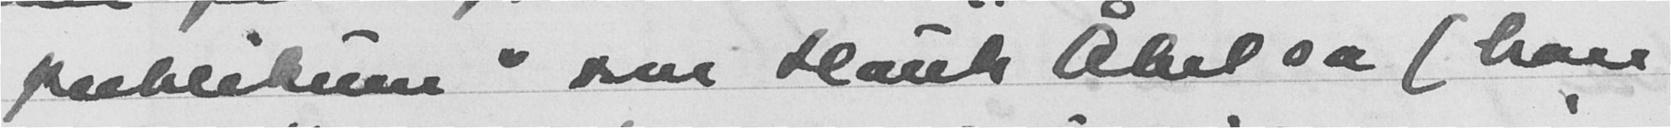publikum" som Hauk Åbel sa (han
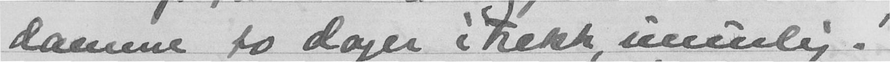damene to dager istrekk, umulig!
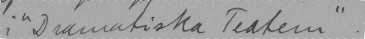i Dramatiska Teatern".
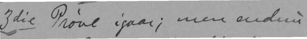3die Prøve igaar; men endnu
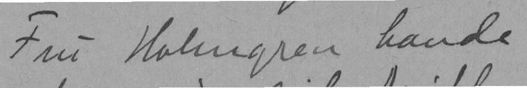Fru Holmgren havde
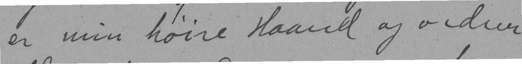er min høire Haand og ordner
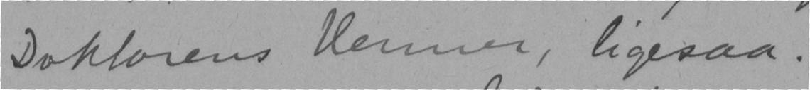Doktorens Venner, ligesaa.
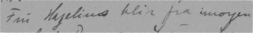Fru Hagelius blir fra imorgen
